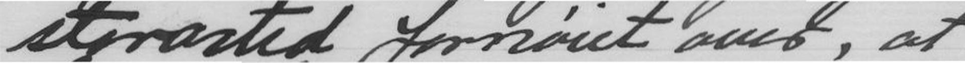storartet fornøiet over, at
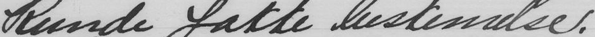kunde fatte bestemelse.
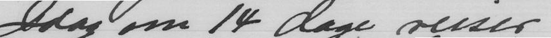Idag om 14 dage reiser
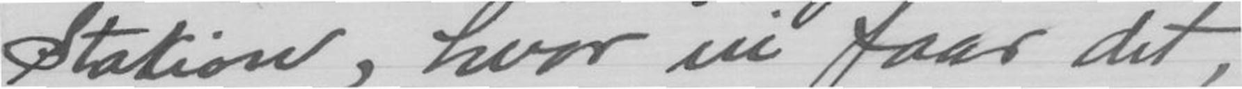Station, hvor vi faar det,
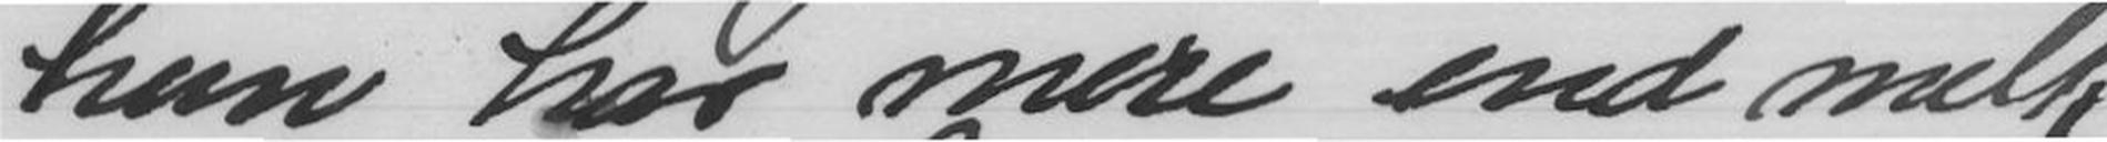hun har mere end melk
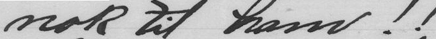nok til ham!!
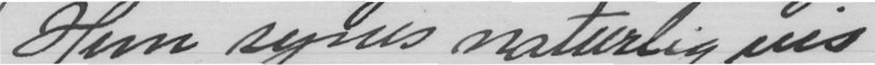Hun synes naturligvis
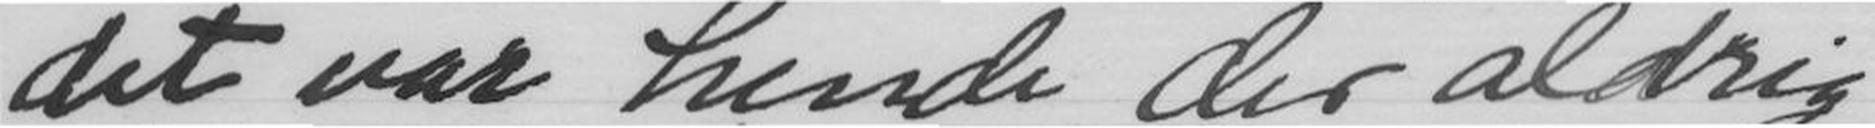det var hende der aldrig
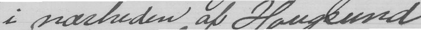i nærheden af Hougsund
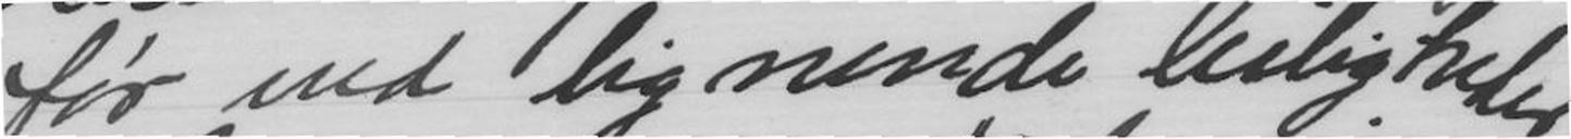før ved lignende leiligheder.
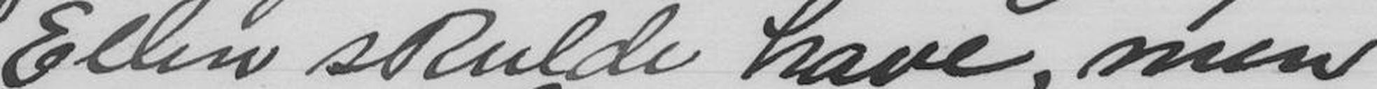Ellen skulde have, men
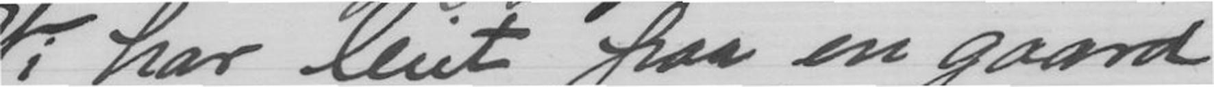Vi har leiet pa en gaard
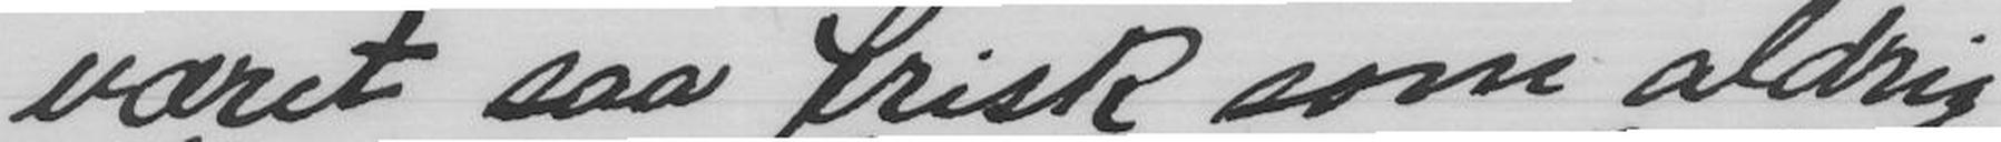været saa frisk som aldrig
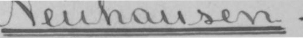Neuhausen.
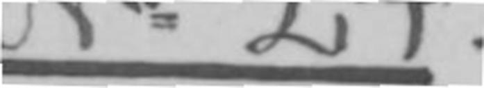No 24.
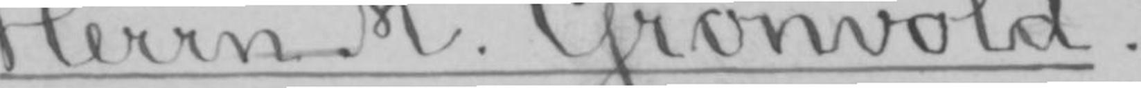Herrn M. Grønvold.
